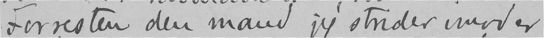Forresten den mand jeg strider imod er
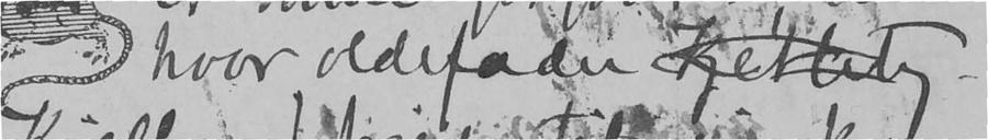hvor oldefader Zetlitz
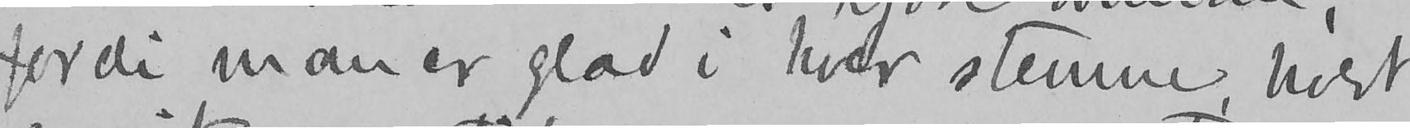fordi man er glad i hver stemme, hvert
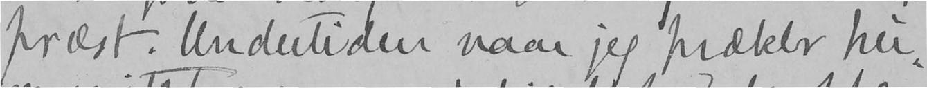præst. Undertiden naar jeg præker hu-
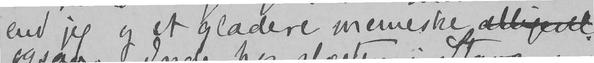end jeg og et gladere menneske alligevel
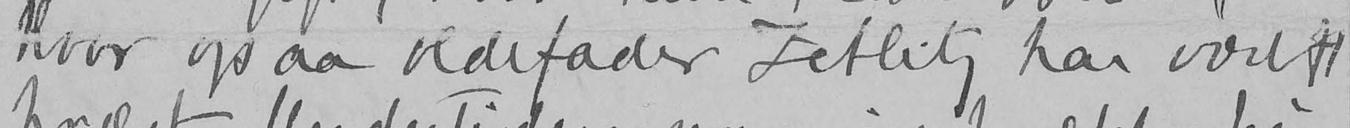hvor ogsaa oldefader Zetlitz har været
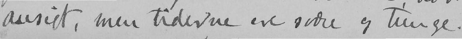ansigt, men tiderne ere svære og tunge.
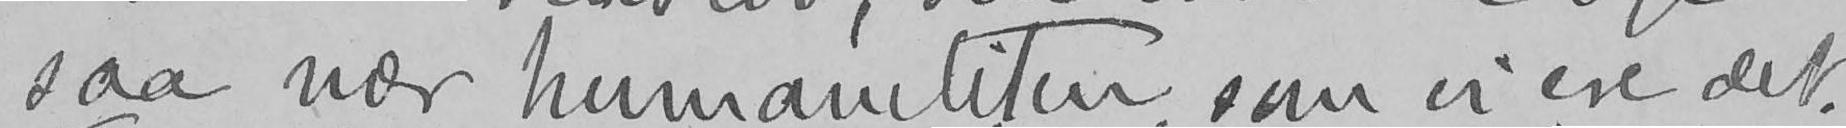saa nær humaniteten, som vi ere det.
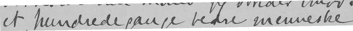et hundrede gange bedre menneske
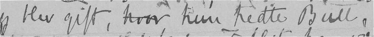og blev gift, hvor hun hedte Bull,
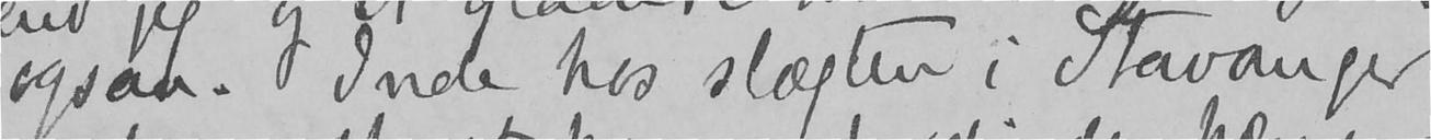ogsaa. Inde hos slægten i Stavanger
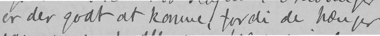er der godt at komme, fordi de hænger
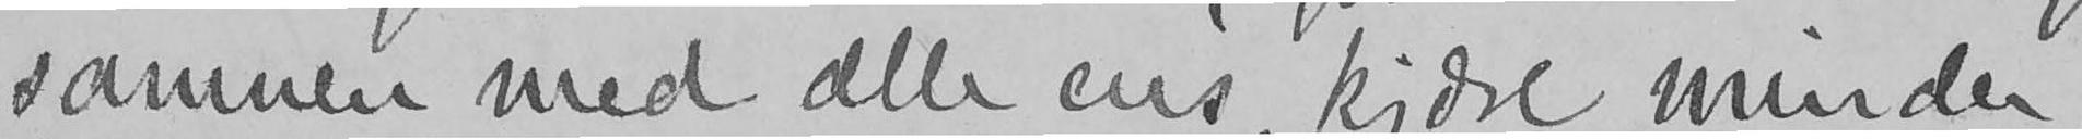sammen med alle ens kjære minder,
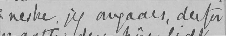neske, jeg omgaaes, derfor
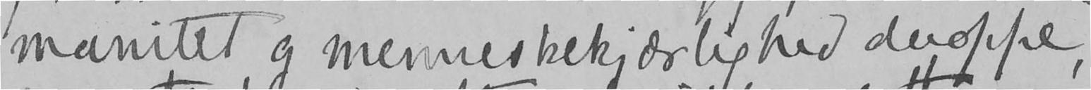manitet og menneskekjærlighed deroppe,
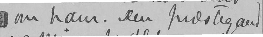om ham. Den præstegaard
